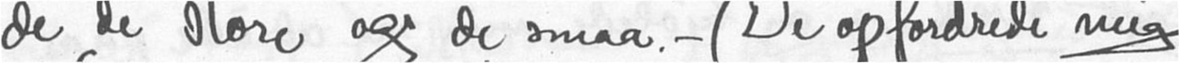de de store og de smaa. - (De opfordrede mig
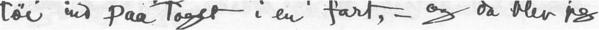tøi ind paa toget i en fart,- og da blev jeg
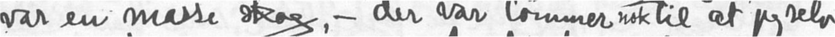var en masse skog, - der var tommer nok til at jeg selv
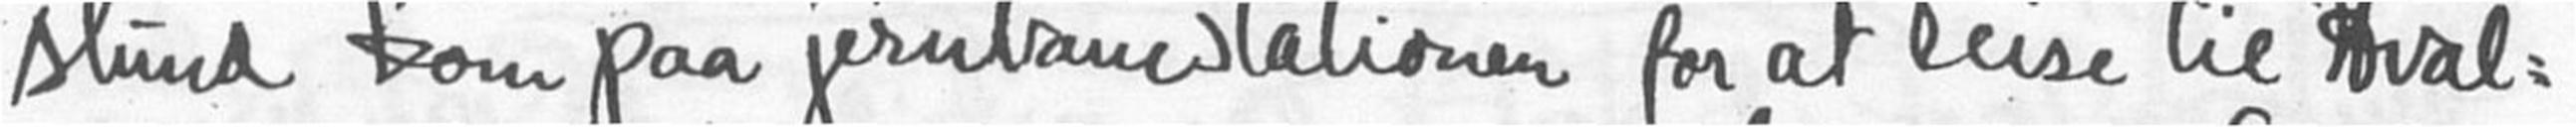stund kom paa jernbanestationen for at reise til Hval-
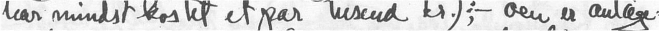har mindst kostet et par tusend kr.) :- øen er antage-
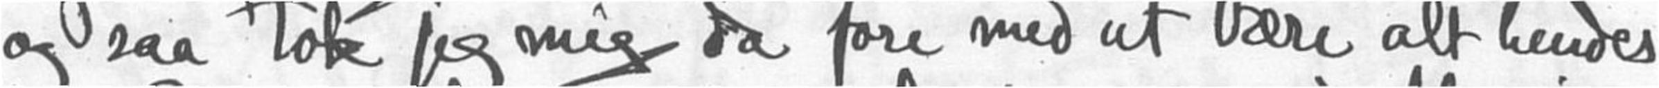og saa tok jeg mig da fore med at bære alt hendes
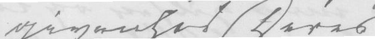givenhed Deres
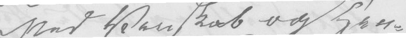Med Venskab og Hen-
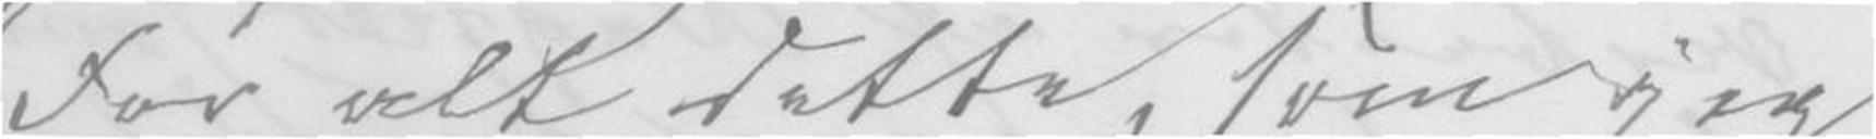For alt dette, som jeg
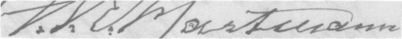J.P.E.Hartmann
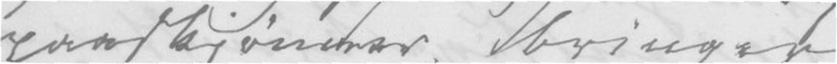paaskjønner, bringer
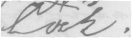Tak.
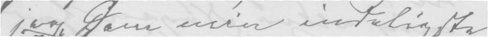jeg Dem min indeligste
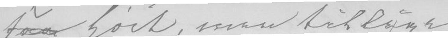saa høit, men tillige
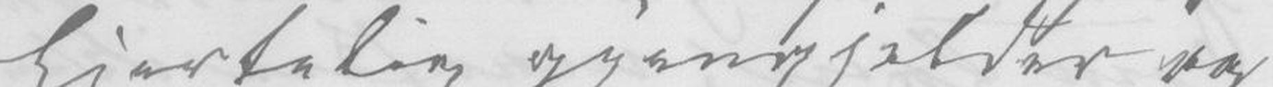hjertelig gjengjelder og
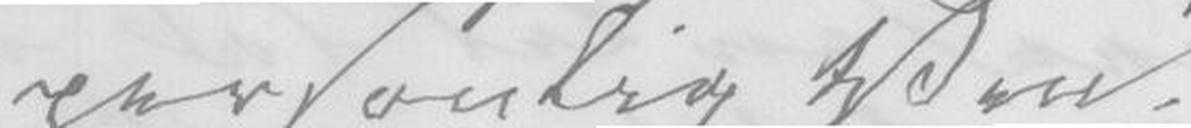personlig Ven,
